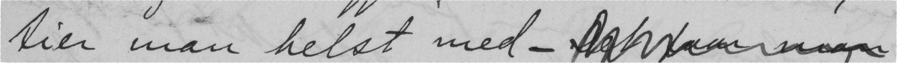tier man helst med -
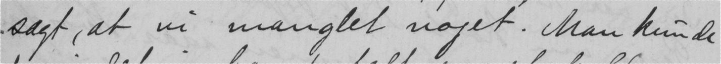sagt, at vi manglet noget. Man kunde
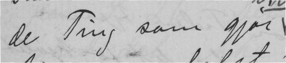de Ting som gjør
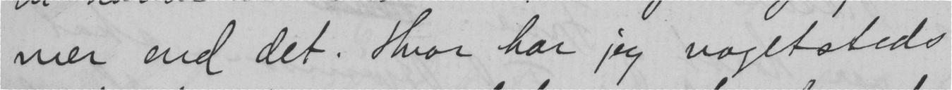mer end det. Hvor har jeg nogetsteds
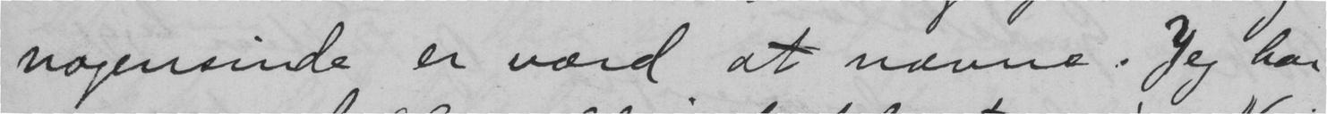nogensinde er værd at nævne. Jeg har
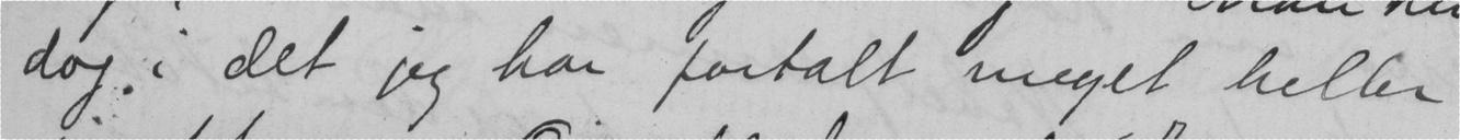dog, i det jeg har fortalt meget heller
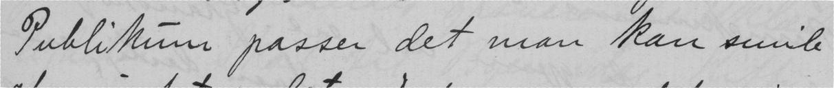Publikum passer det man kan smile
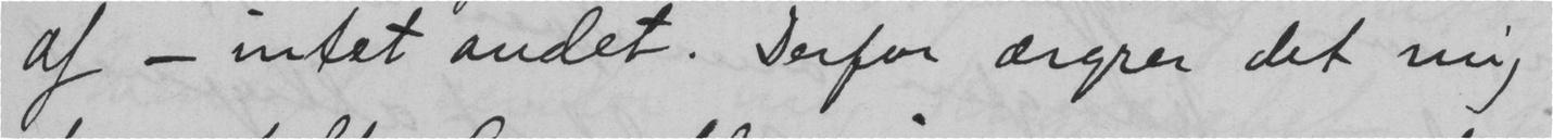af - intet andet. Derfor ærgrer det mig
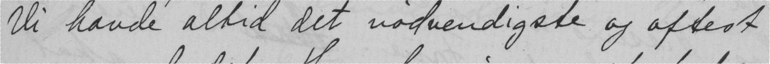Vi havde altid det nødvendigste og oftest
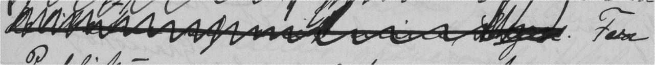For
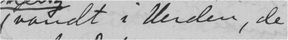vondt i verden, de
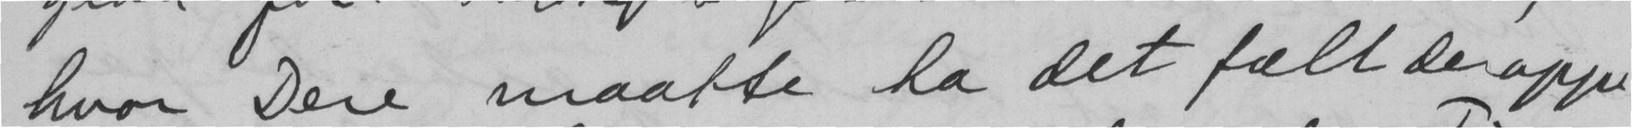hvor Dere maatte ha det fælt deroppe
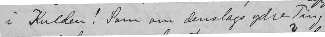i Kulden! Som om denslags ydre Ting
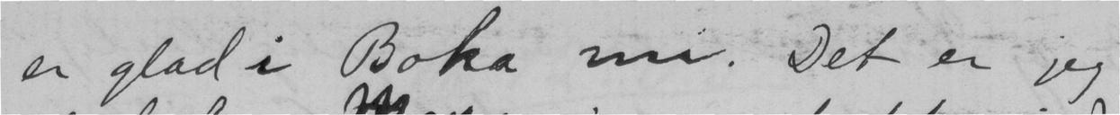er glad i Boka mi. Det er jeg
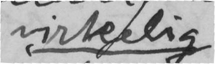virkelig
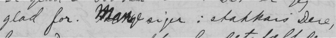glad for.  siger: stakkars Dere,
